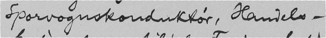Sporvognskonduktør, Handels-
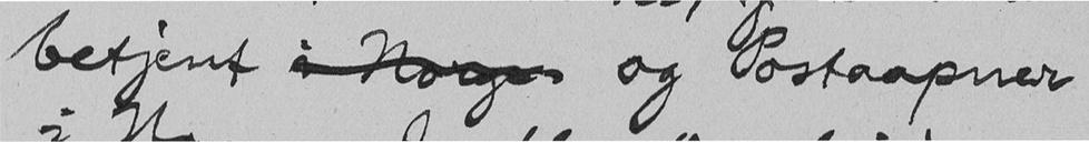betjent i Norge og Postaapner
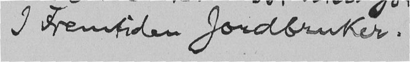I Fremtiden Jordbruker.
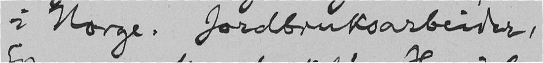i Norge. Jordbruksarbeider,
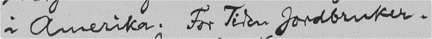i Amerika. For Tiden Jordbruker.
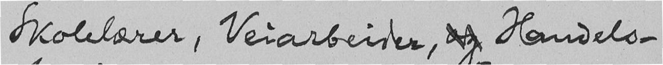Skolelærer, Veiarbeider, og Handels-
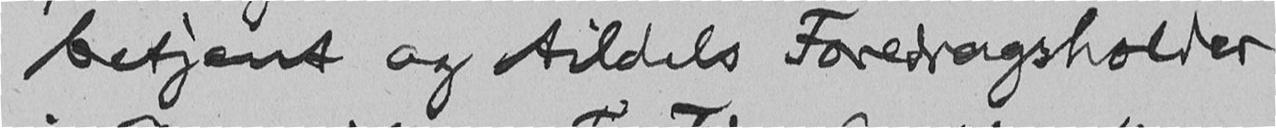betjent og tildels Foredragsholder
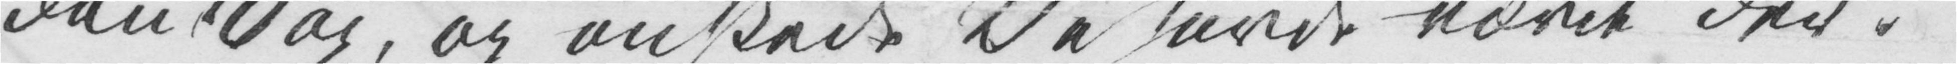den Dag, og ønskede De havde været der!
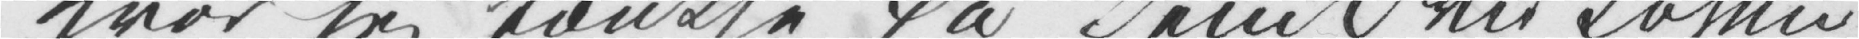Hvor jeg tænkte på Dem Fru Ibsen
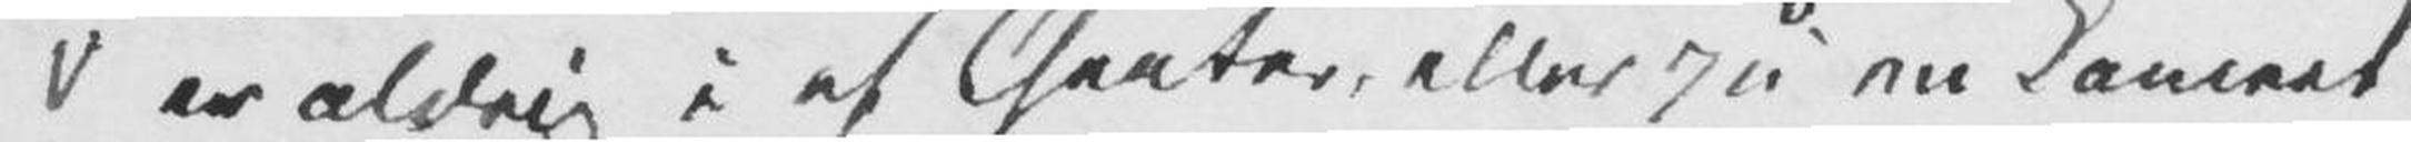er aldrig i et Theater, eller på en Koncert
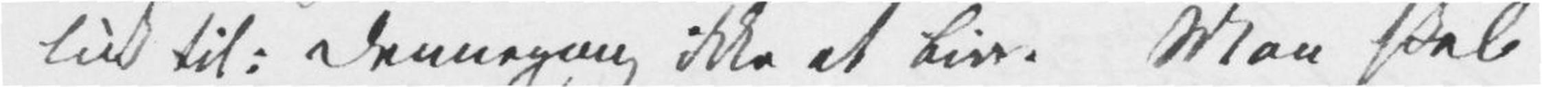lidt til: Dennegang ikke et Liv. Man skal
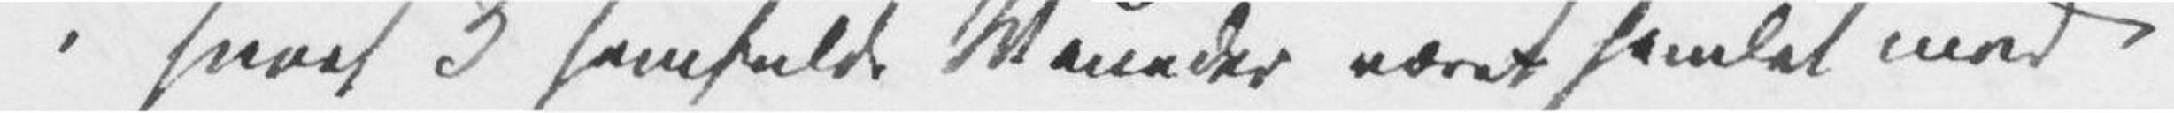i snart 3 samfulde Måneder været samlet med
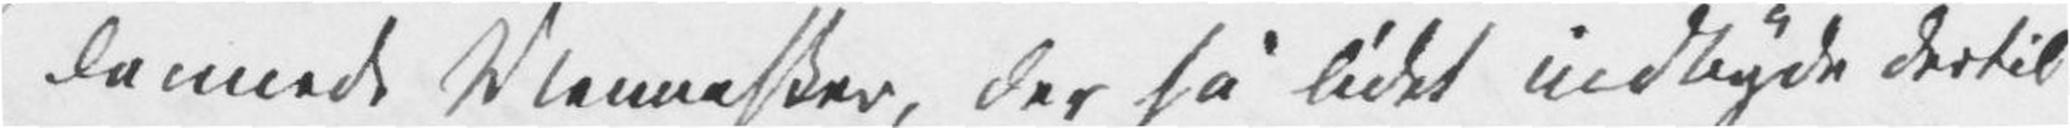dannede Mennesker, der så lidet indbyde dertil
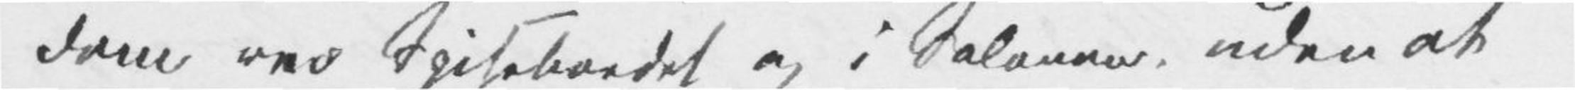dem ved Spisebordet og i Salonen, uden at
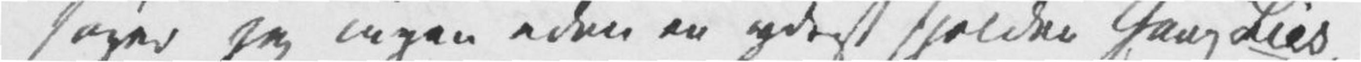søger jeg ingen uden en yderst sjelden gang Lies,
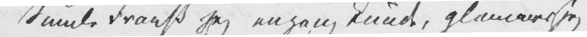Smule Fransk jeg engang kunde, glemmer jeg
